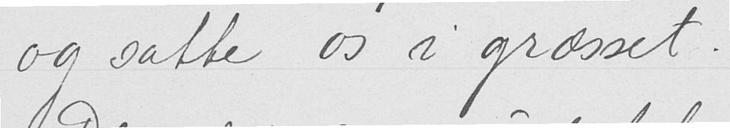og satte os i græsset.
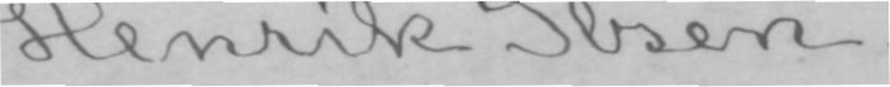Henrik Ibsen.
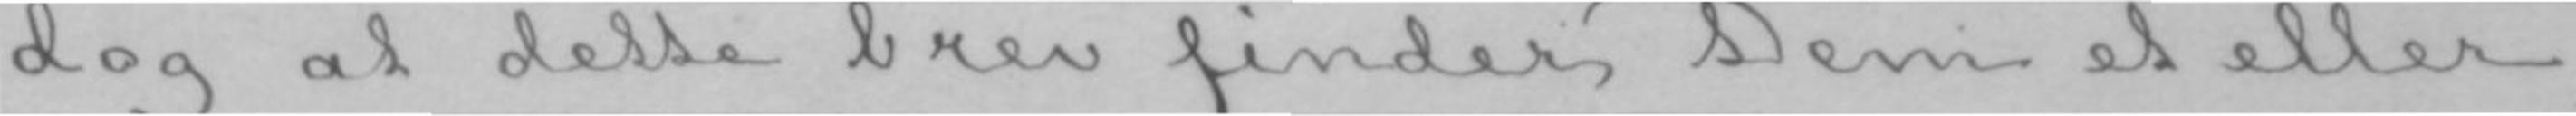dog at dette brev finder Dem et eller
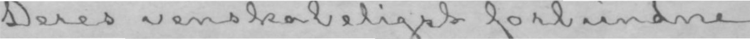Deres venskabeligst forbundne
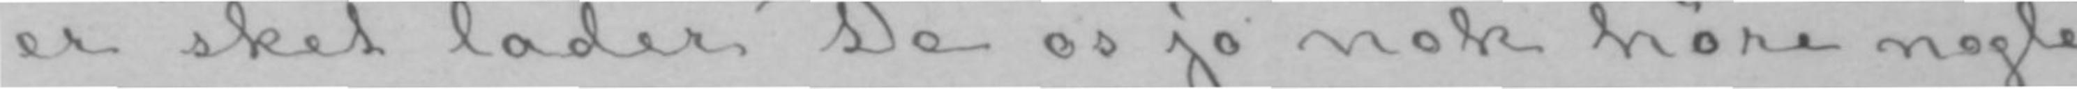er sket lader De os jo nok høre nogle
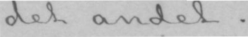det andet.
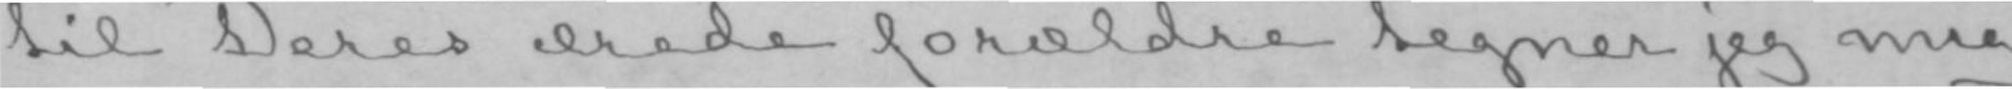til Deres ærede forældre tegner jeg mig
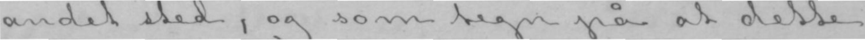andet sted, og som tegn på at dette
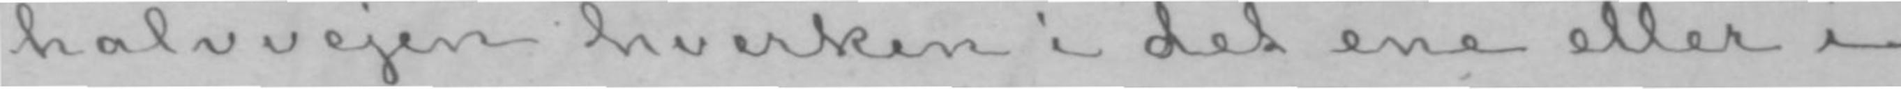halvvejen hverken i det ene eller i
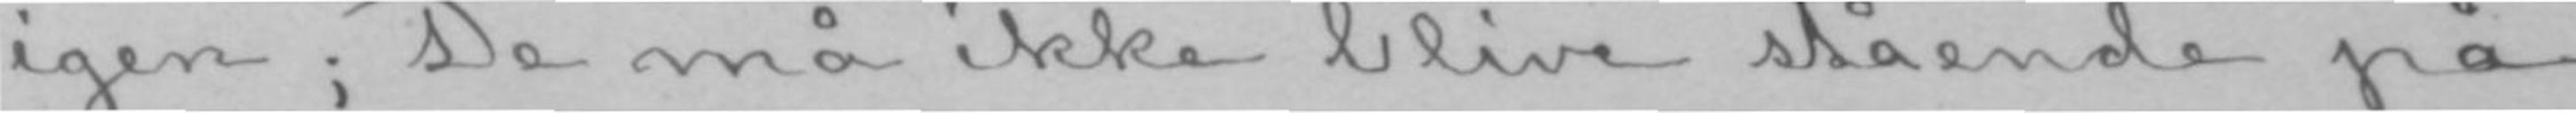igen; De må ikke blive stående på
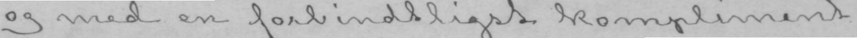og med en forbindtligst kompliment
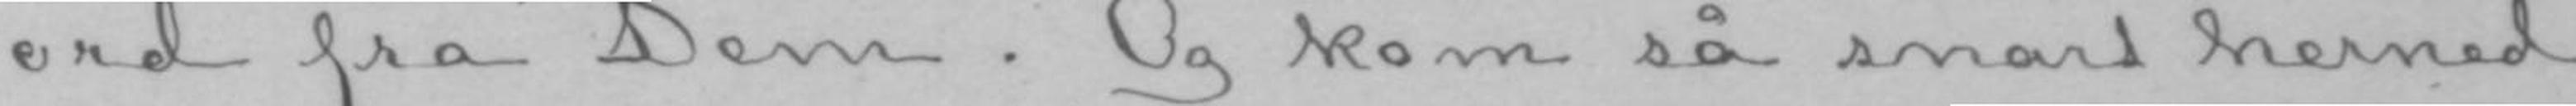ord fra Dem. Og kom så snart herned
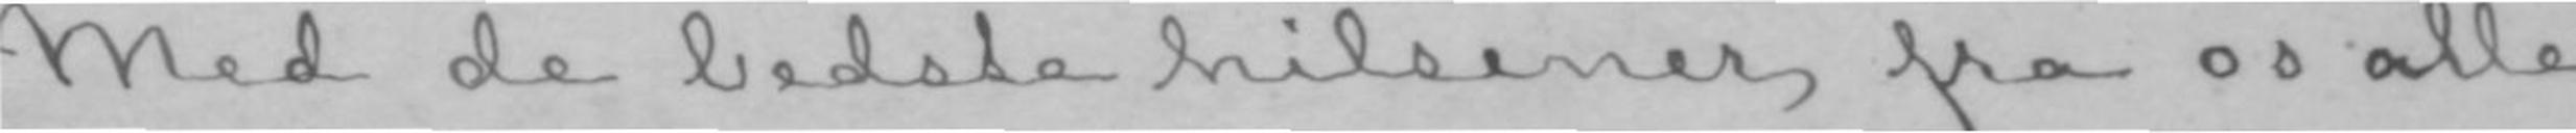Med de bedste hilsener fra os alle
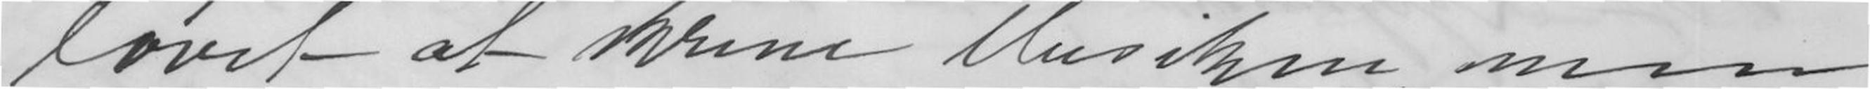lovet at skrive Musiken, men
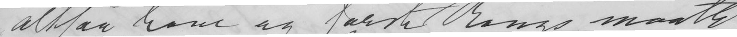altsaa have og første Rangs maatte
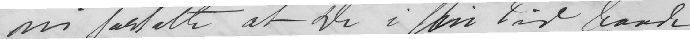vi fortalte at De i sin Tid havde
